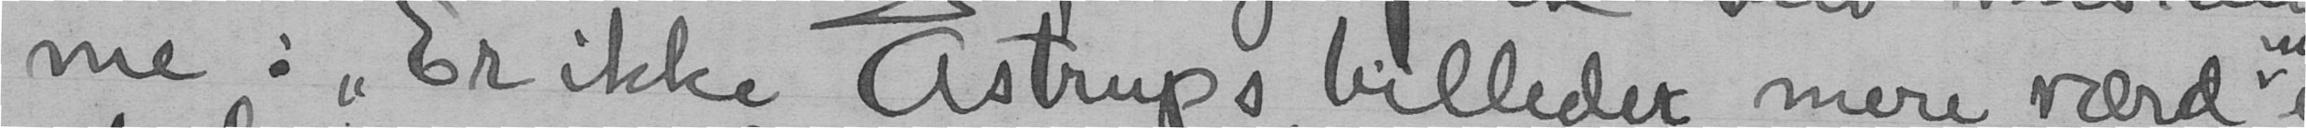me: "Er ikke Astrups billeder" mere værd,
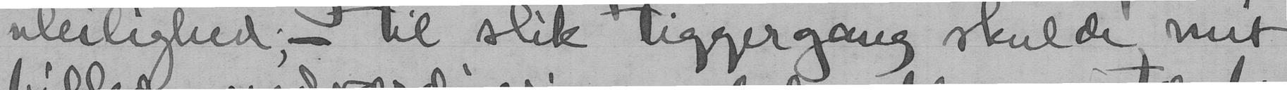uleilighed, - til slik tiggergang skulde mit
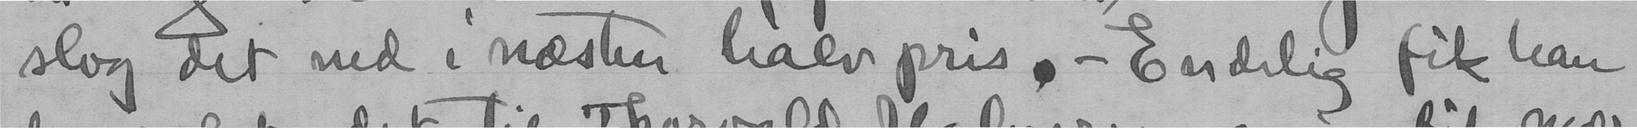slog det ned i næsten halv pris, - Endelig fik han
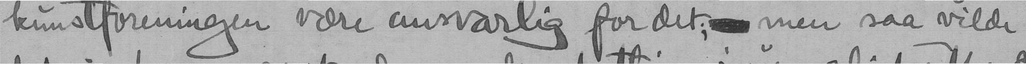kunstforeningen bære ansvarlig for det, - men saa vilde
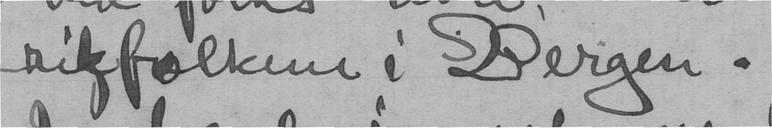rikfolkene i Bergen.
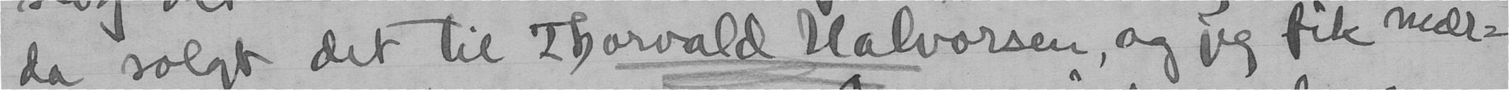da solgt det til Thorvald Halvorsen, og jeg fik mær-
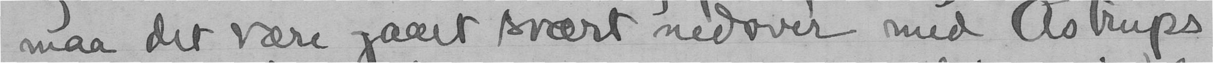maa det være gaaet svært nedover med Astrups
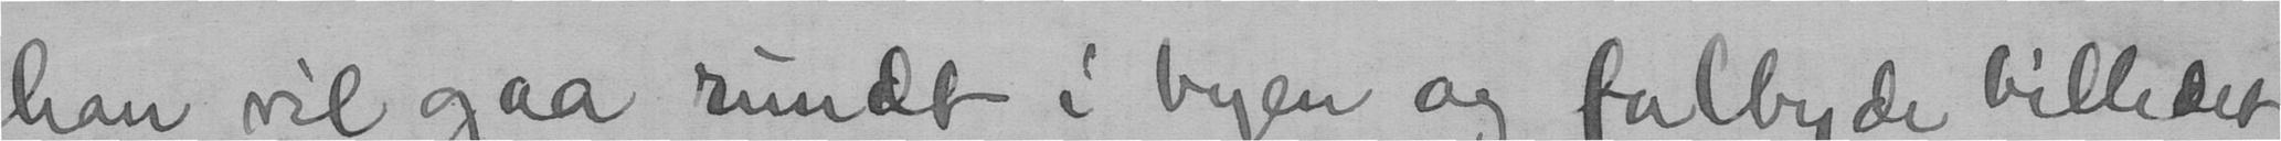han vil gaa rundt i byen og falbyde billedet
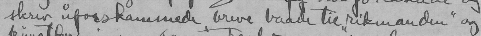skrev uforskammede breve baade til "rikmanden" og
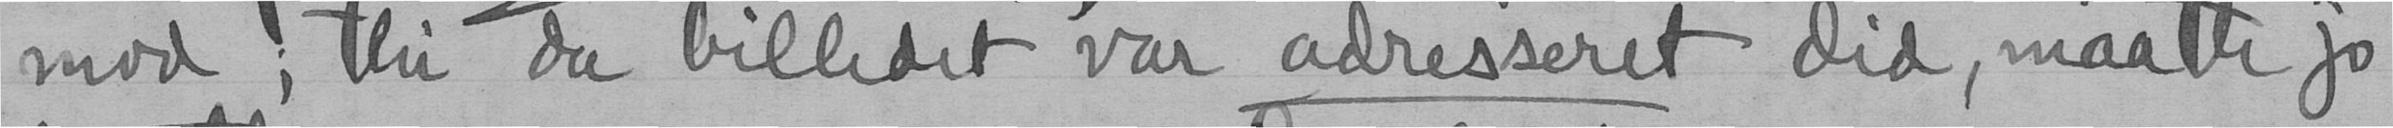mod; thi da billedet var adresseret did, maatte jo
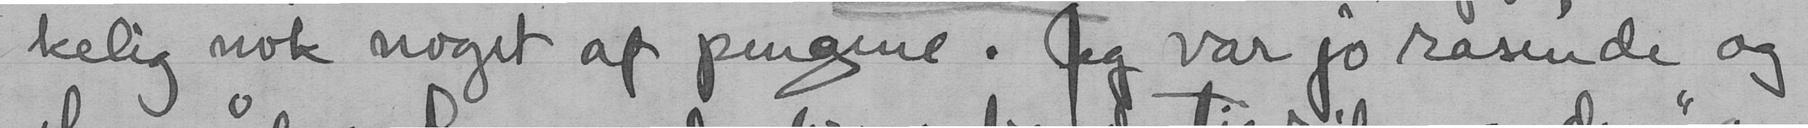kelig nok noget af pengene. Jeg var jo rasende og
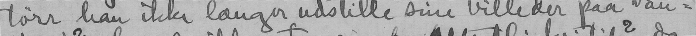tørr han ikke længer udstille sine billeder paa van-
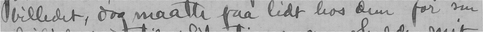billedet, dog maatte faa lidt hos dem for sin
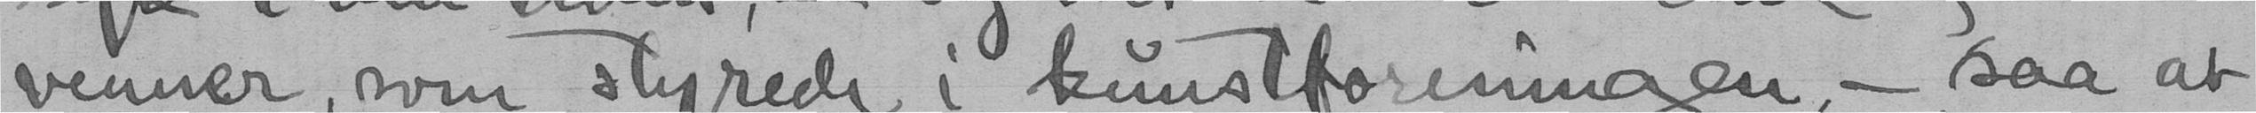venner, som styrede i kunstforeningen, - saa at
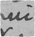nu
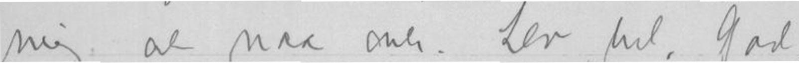mig at naa over. Lev vel. God,
